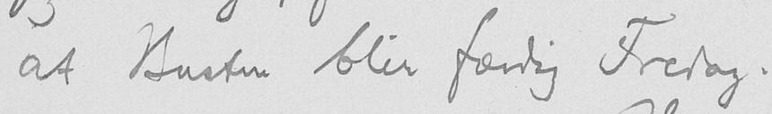af Busten blir færdig Fredag.
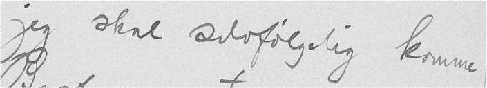jeg skal selvfølgelig komme
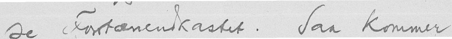se Fontæneudkastet. Saa kommer
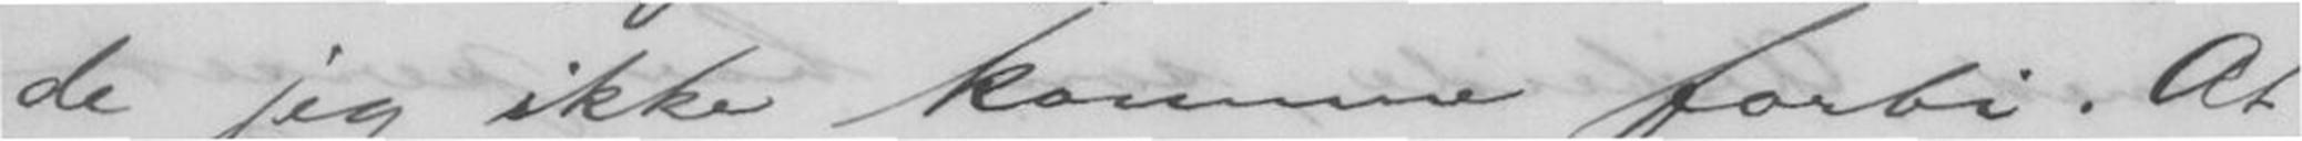de jeg ikke komme forbi. At
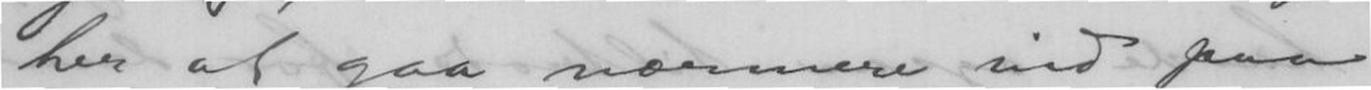her at gaa nærmere ind paa
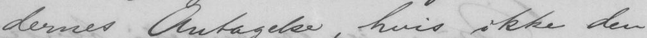dernes Antagelse, hvis ikke den
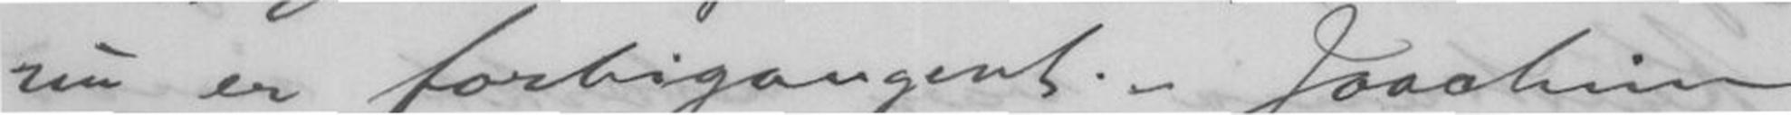nu er forbigangent. - Joachim
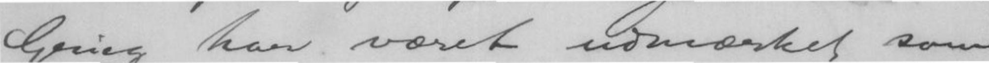Grieg har været udmærket som
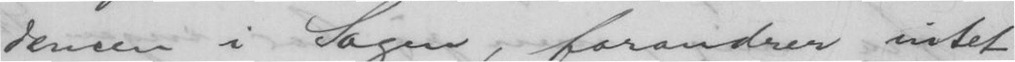densen i Sagen, forandrer intet
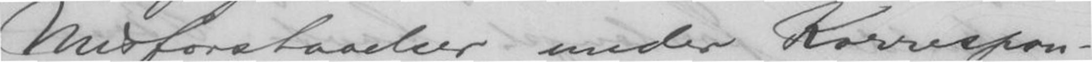Misforstaaelser under Korrespon-
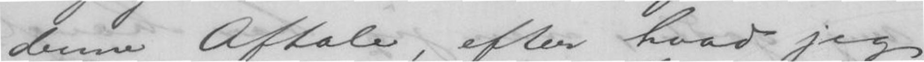denne Aftale, efter hvad jeg
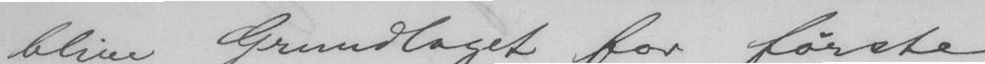blive Grundlaget for første
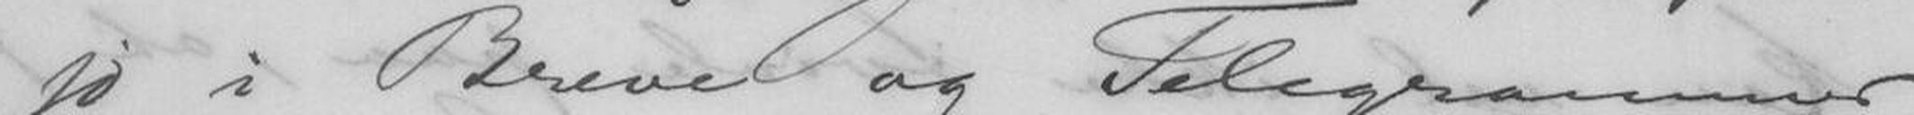jo i Breve og Telegrammer
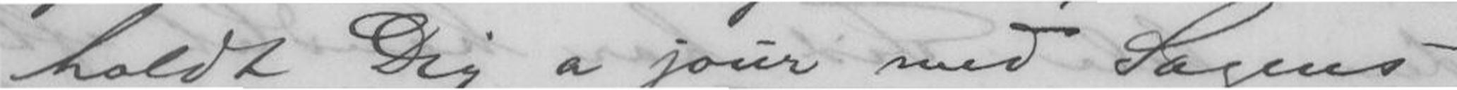holdt Dig a jour med Sagens
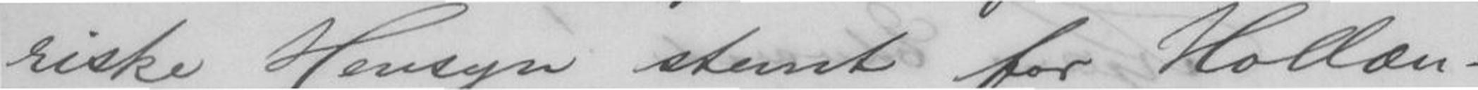riske Hensyn stemt for Hollæn-
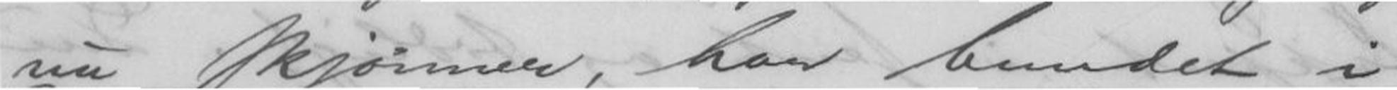nu skjønner, har bundet i
